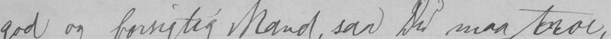god og forsigtig Mand, saa Du maa troe,
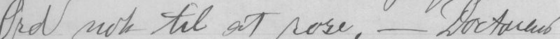Ord nok til at rose, - Doctorens
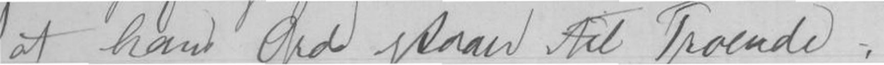at hans Ord staaer til Troende.
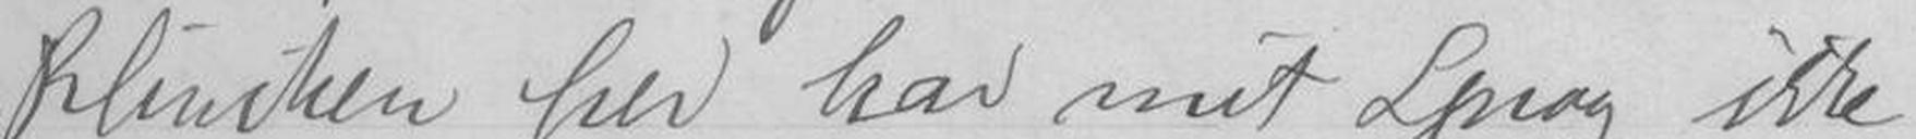Klinicken her har mig Sprog ikke
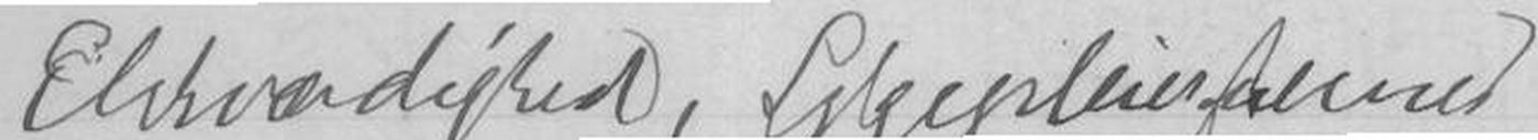Elskværdighed, Sygepleierskenes
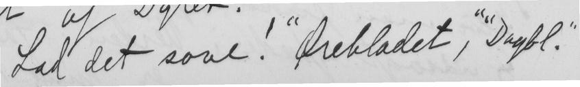Lad det sove! "Ørebladet", "Dagbl."
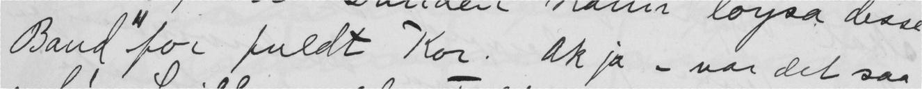Band" for fuldt Kor. Ak ja - var det saa
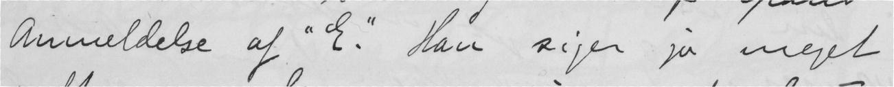Anmeldelse af "E." Han siger jo meget
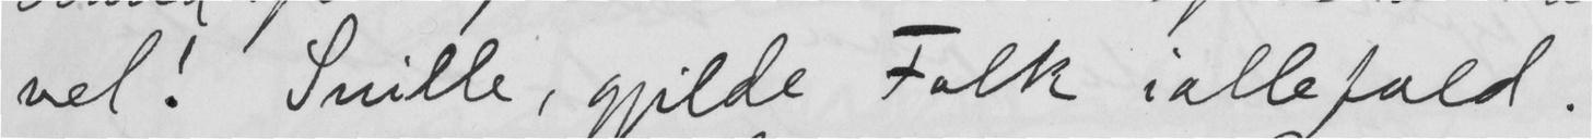vel! Snille, gjilde Folk iallefald.
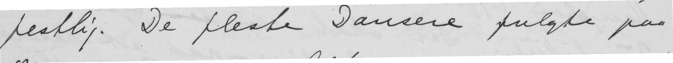festlig. De fleste Dansere fulgte paa
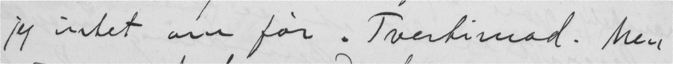Jeg intet om for. Tvertimod. Men
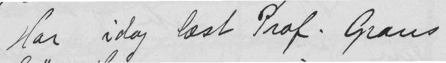Har idag læst Prof. Grans
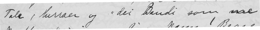Tale, Hurraer og " dei Bandi som me
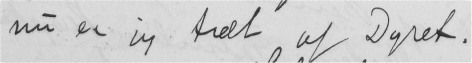nu er jeg træt af Dyret.
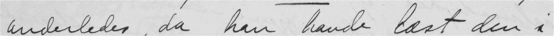anderledes, da han havde læst den i
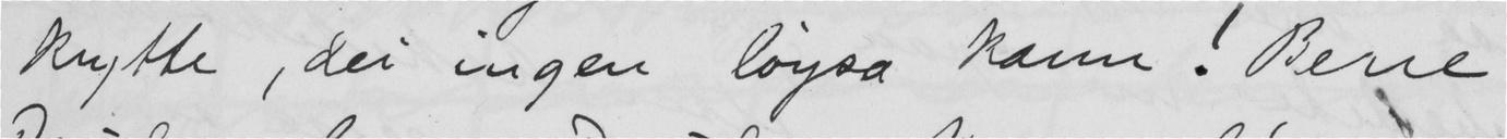knytte, dei ingen løysa kann! Berre
